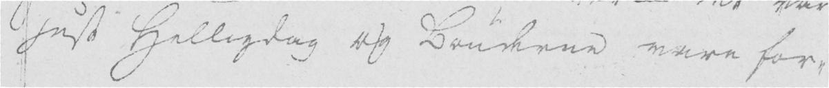just Helligdag og Bønderne vare for-
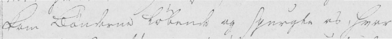kom Bønderne løbende og spurgte os hvor
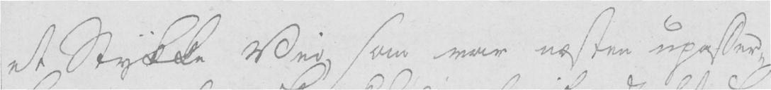et stykke Vej, som var næsten upasser-
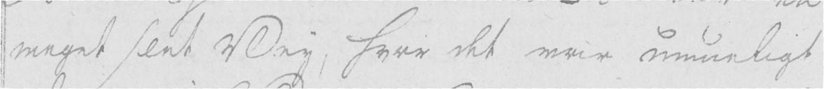meget slet Vey, hvor det var umueligt
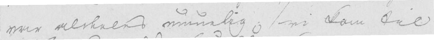var aldeles umuelig; vi kom til
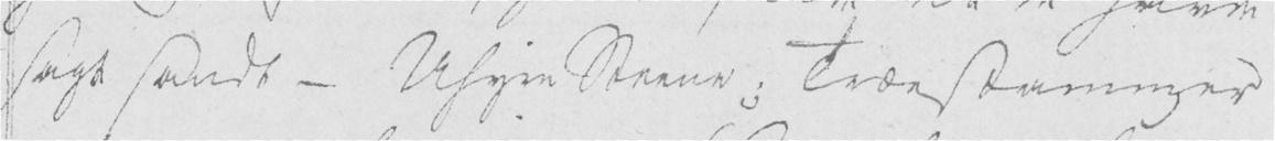sagt sandt - Uhyie Steene; Træestammer
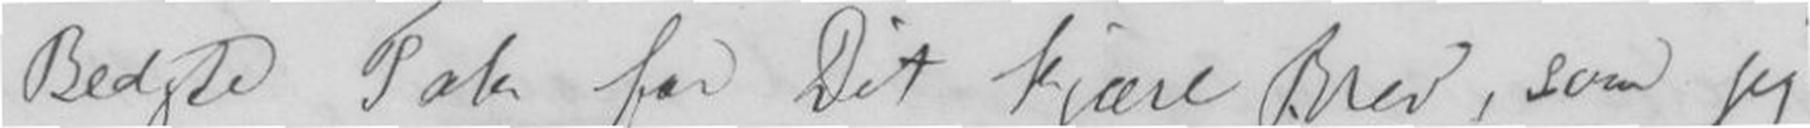Bedste Tak for Dit kjære Brev, som jeg
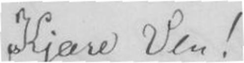Kjære Ven!
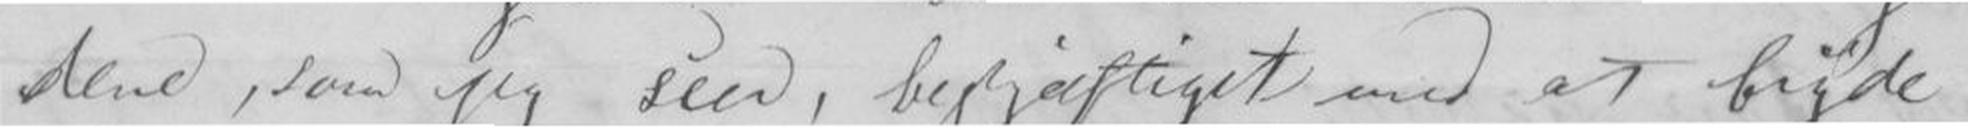lene, som jeg seer, beskjæftiget med at bryde
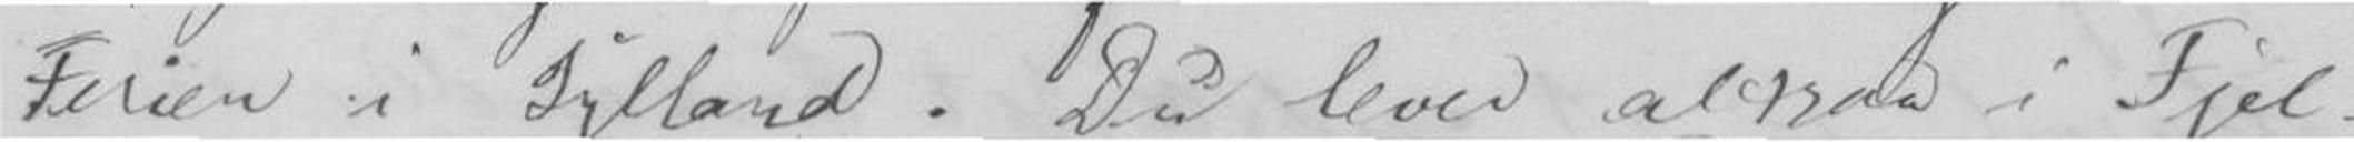Ferien i Jylland. Du lever altsaa i Fjel-
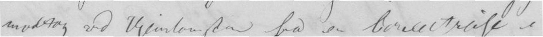modtog ved Hjemkomsten fra en Concertreise i
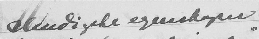elendigste egenskaper
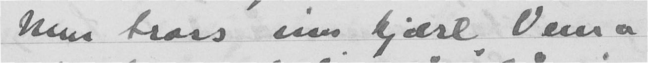Men trass sin kjære Vema
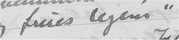og frues legem."
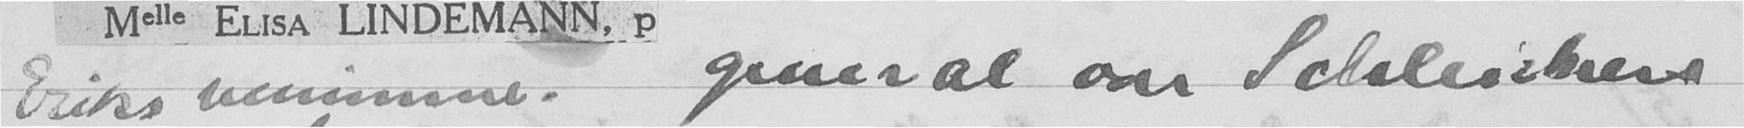Eriks venninne general omr Schleichsess
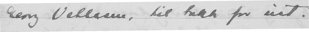Georg Vetlesen, til takk for int.
